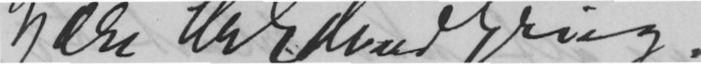Kjære Hr Edvard Grieg!
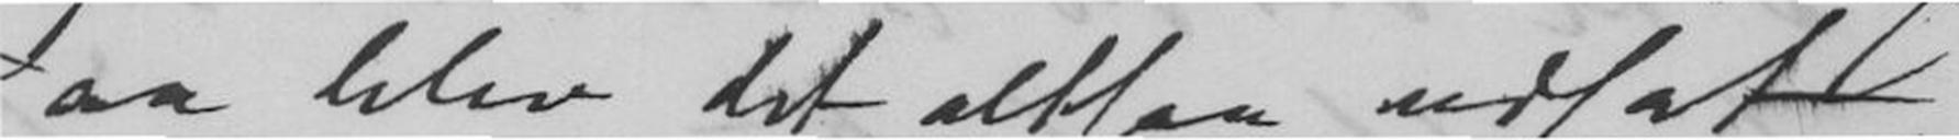Saa blev det altsaa udsat.
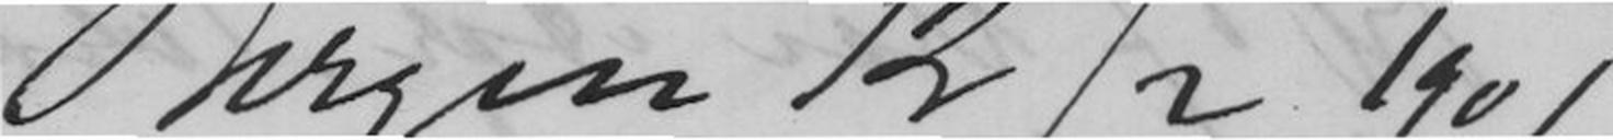Bergen 12/2 1901
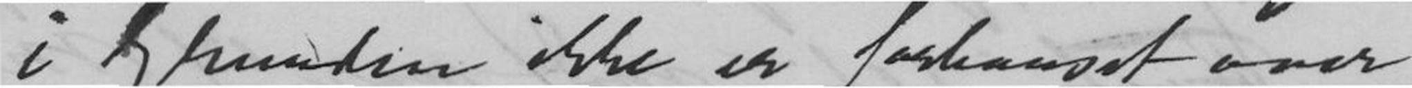i grunden ikke er forbauset over
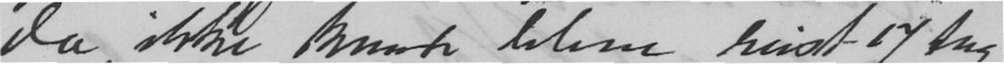da ikke kunde blive reist 17 Aug.
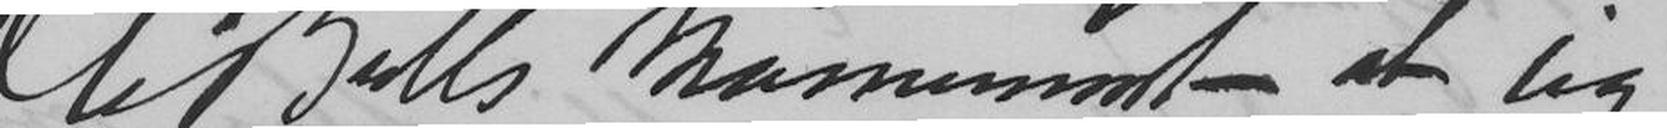Ole Bulls Monument at jeg
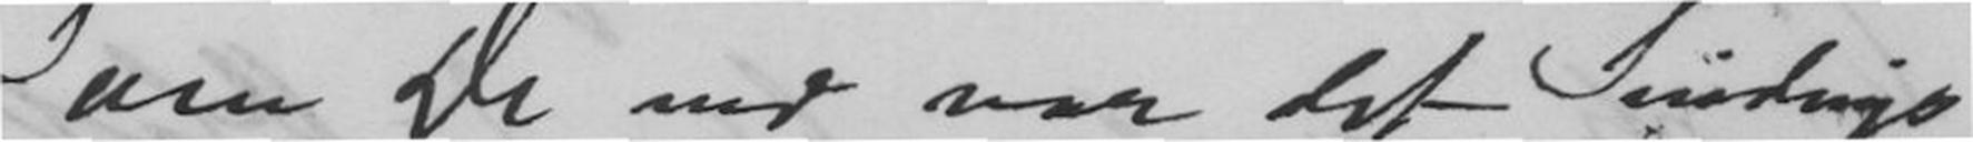Som De ved var det Sindings
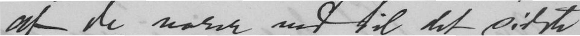at de varer ved til det sidste.
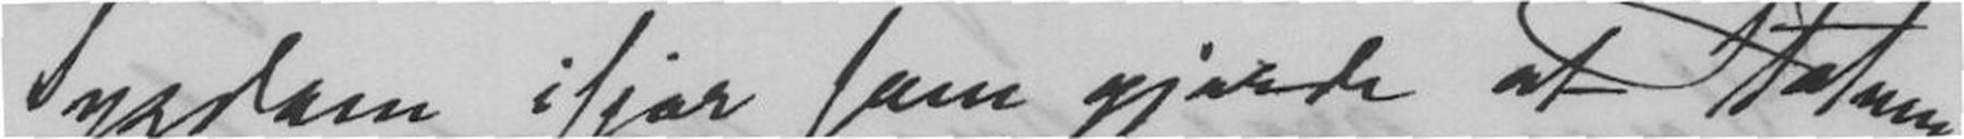Sygdom ifjor som gjorde at Statuen
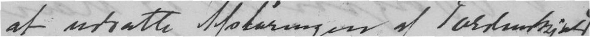at udsætte Afslørningen af Tordenskjold
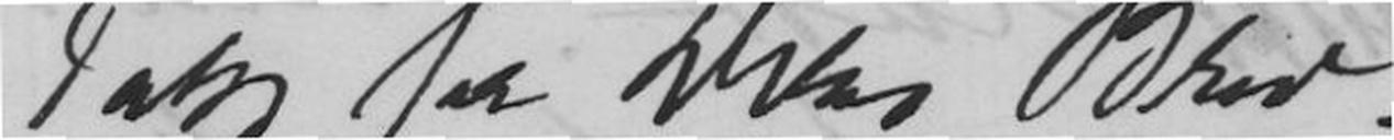Takk for Deres Brev.
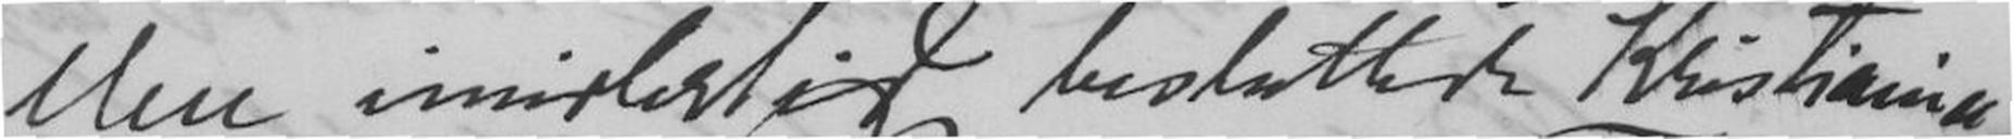Men imidlertid besluttede Kristiania
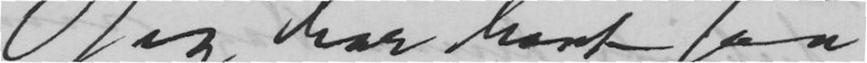Jag har havt saa
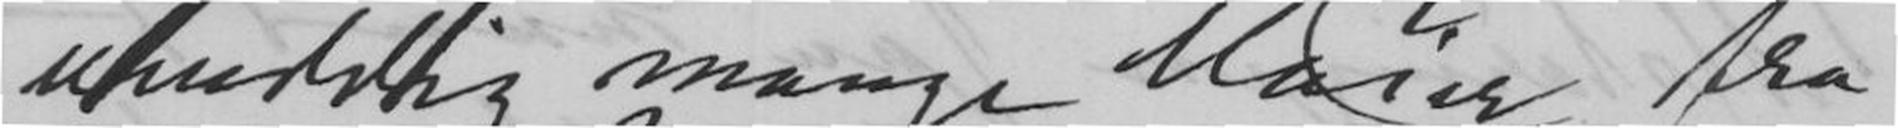uendelig mange Møier fra
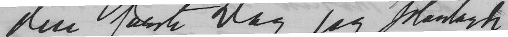den første Dag jeg planlagde
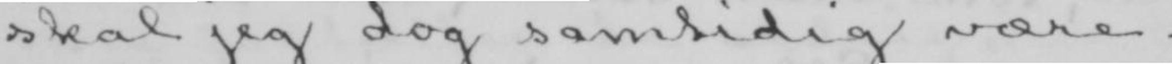skal jeg dog samtidig være.
